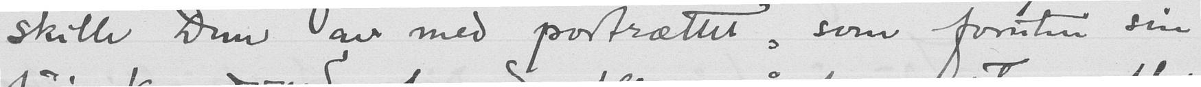skille Dem av med portrættet, som foruten sin
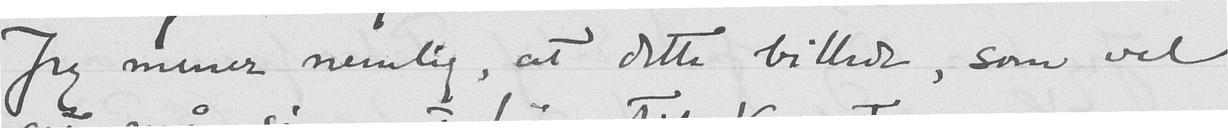Jeg mener nemlig, at dette billede, som vel
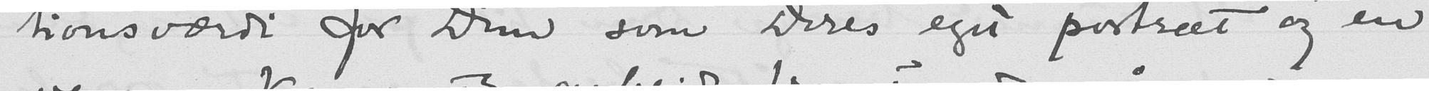tionsværdi for Dem som Deres eget portræt og en
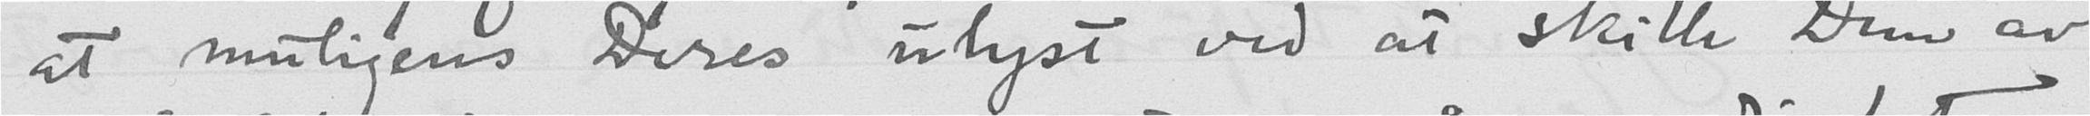at muligens Deres ulyst ved at skille Dem av
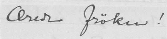Ærede frøken!
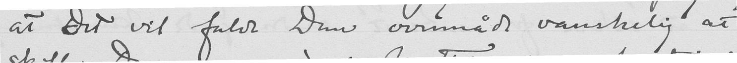at Det vil falde Dem overmåde vanskelig at
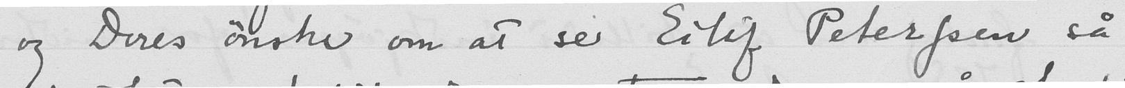og Deres ønske om at se Eilif Peterssen så
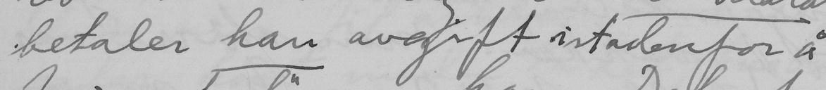betaler han avgift istadenfor å
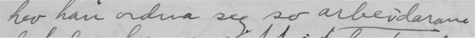hev han ordna seg so arbeidarane
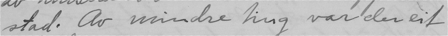stad. Av mindre ting var der eit
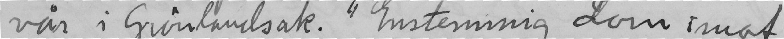vår i Grönlandsak. "Einstemmig dom imot
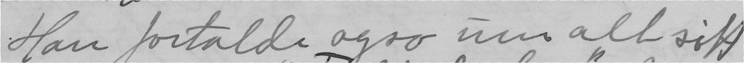Han fortalde ogso um alt sitt
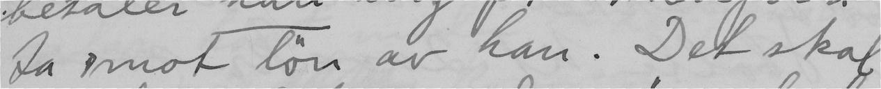ta imot lön av han. Det skal
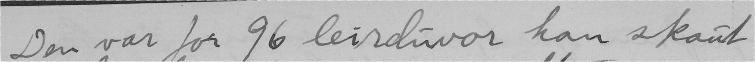Den var for 96 leirduvor han skaut
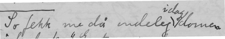So fekk me då endeleg idag domen
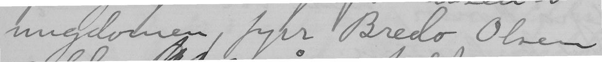ungdomen, fyrr Bredo Olsen
